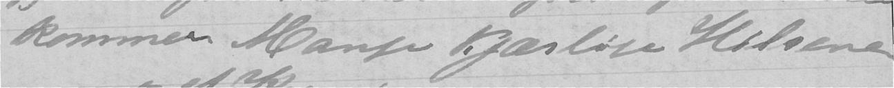kommer. Mange kjærlige Hilsener
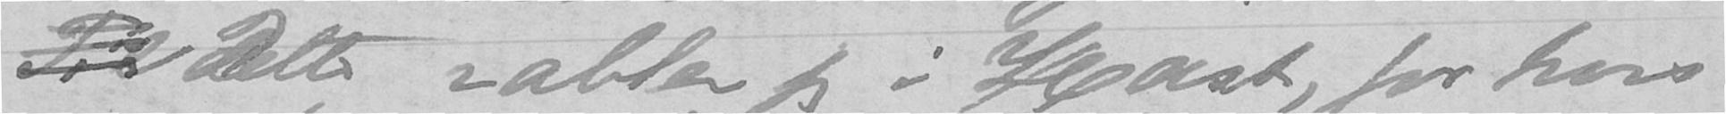Til Dette rabler jeg i Hast, for hvis
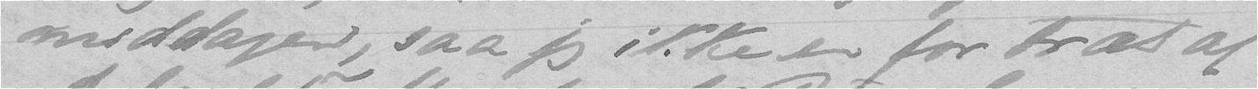middagen, saa jeg ikke er for træt af
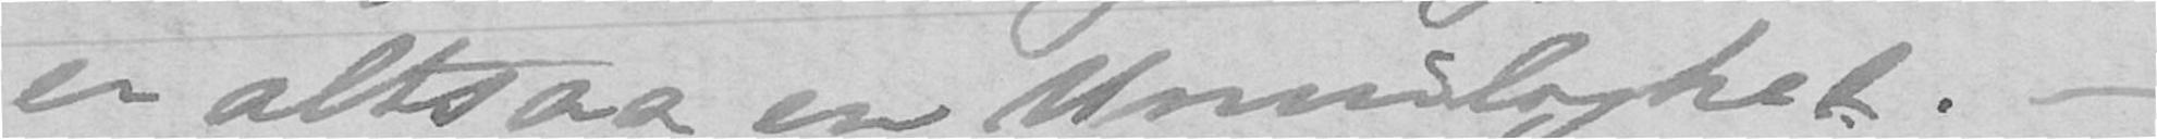er altsaa en Umulighet. -
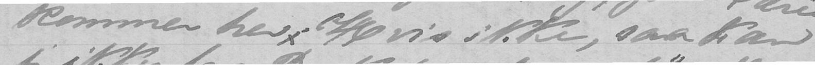kommer her,. Hvis ikke, saa kan
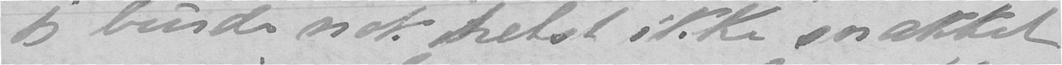jeg burde nok helst ikke snakket
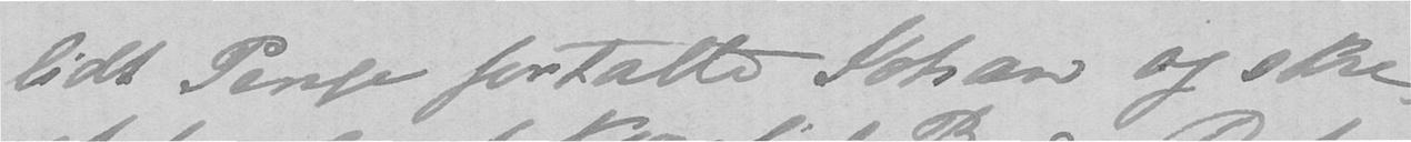lidt Penge fortalte Johan og skre-
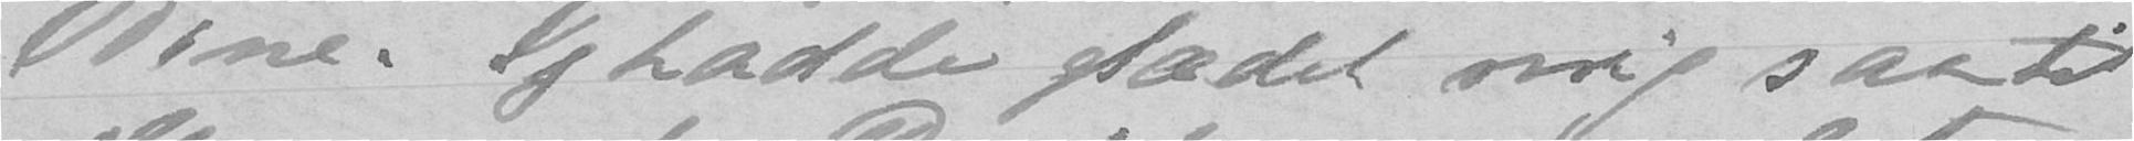Dine. Jeg hadde glædet mig saa til
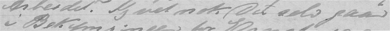Arbeidet? Jeg vet nok Du selv gaar
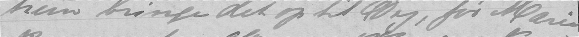hun bringe det op til Dig, før Marie
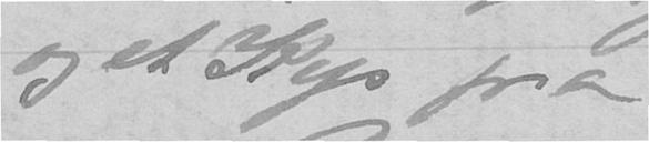og et Kys fra
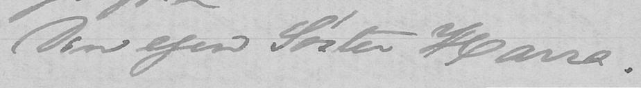Din egen Søster Harra.
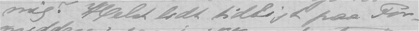mig? Helst lidt tidligt paa For-
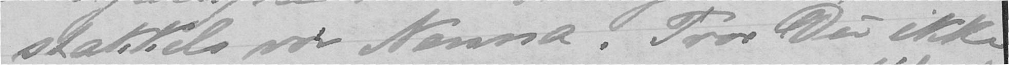stakkels vor Nanna. Tror Du ikke,
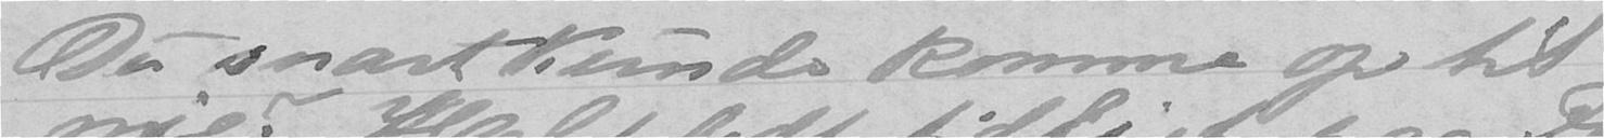Du snart kunde komme op til
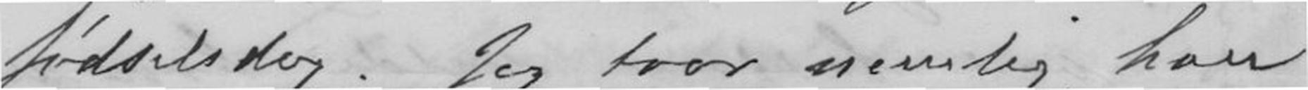fødselsdag. Jeg tror nemlig, han
